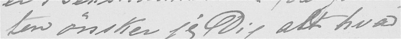ten ønsker jeg Dig alt hvad
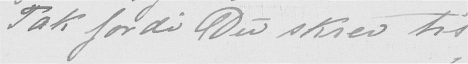Tak fordi Du skrev til
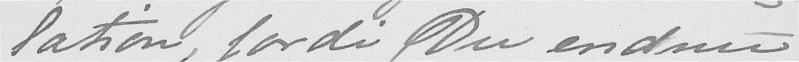lation, fordi Du endnu
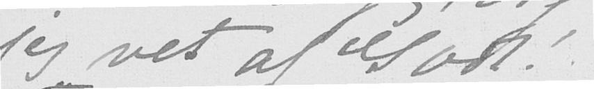jeg vet af Godt!
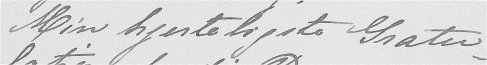Min hjerteligste Gratu-
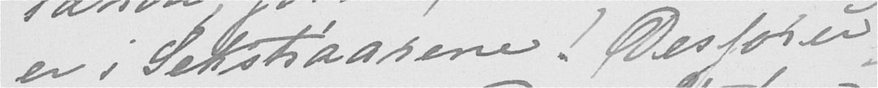er i Sekstiaarene! Desforu-
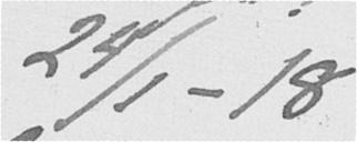28/1-18.
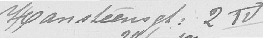Hansteensgt. 2 IV
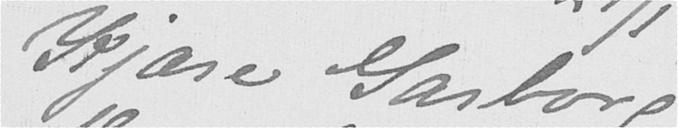Kjære Garborg
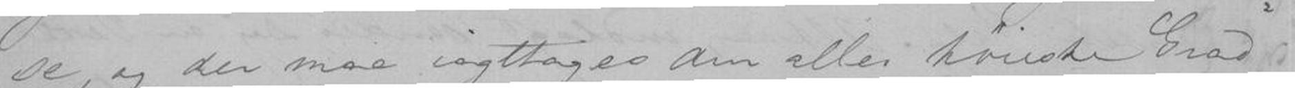se, og der maa iagttages den aller høieste Grad
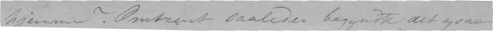hjemme? Omtrent saaledes begyndte det ogsaa
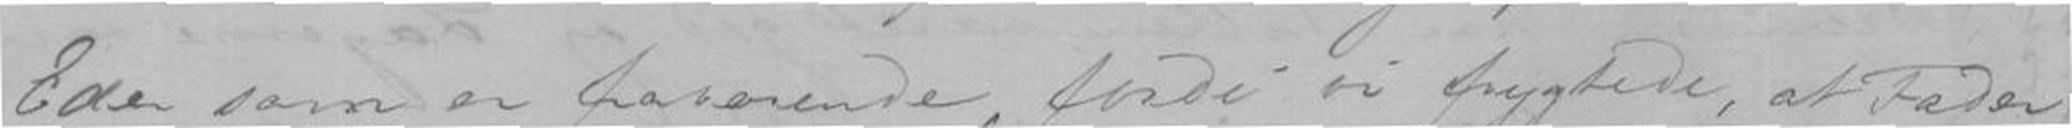Eder som er fraværende, fordi vi frygtede, at Fader
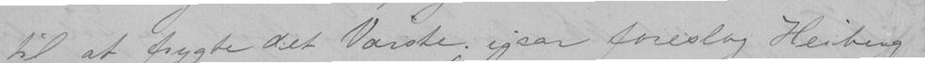til at frygte det Værste, igaar foreslog Heiberg
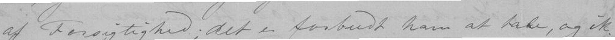af Forsigtighed; det er forbudt ham at tale, og ik-
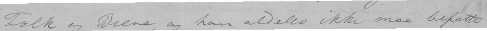Folk og Breve, og han aldeles ikke maa befatte
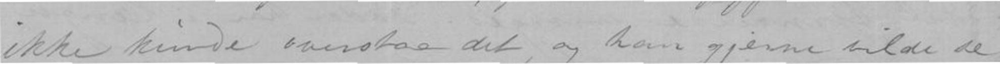ikke kunde overstaa det, og ham gjerne vilde se
